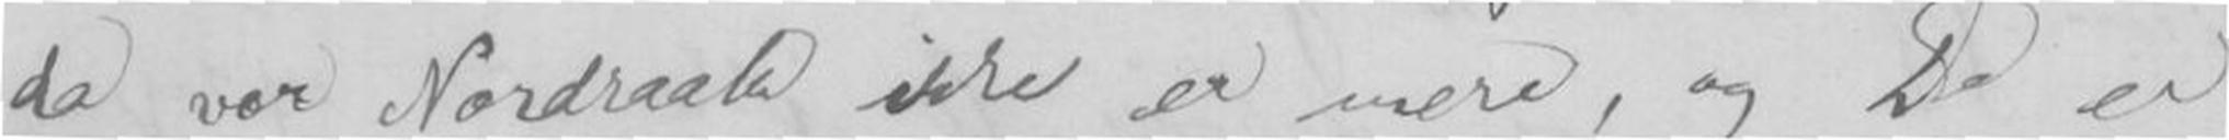da var Nordraak ikke er mere, og De er
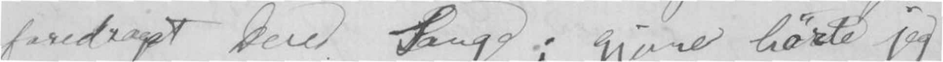foredraget Deres Sange; gjerne hørte jeg
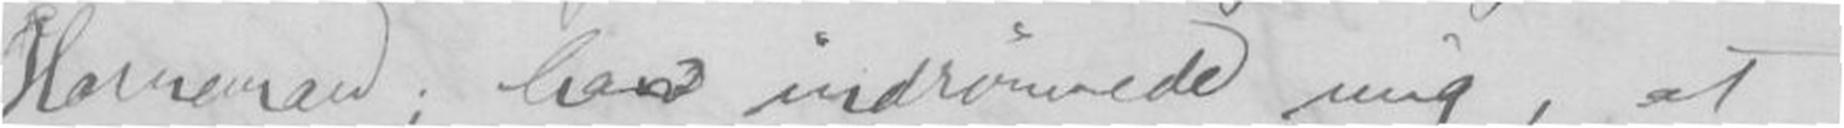Hornemand; han indrømmede mig, at
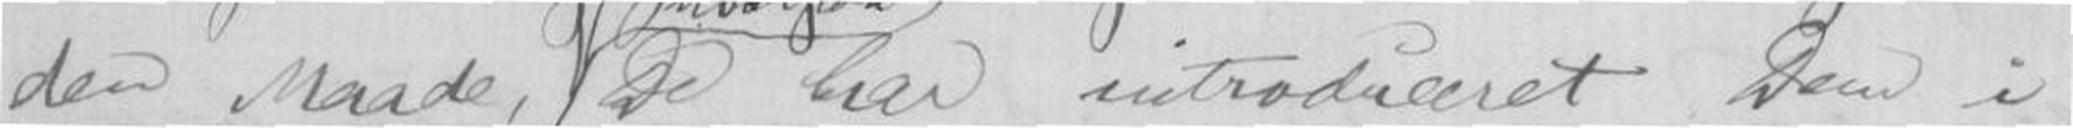den Maade, de har introducerete Dem i
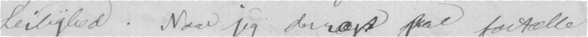Leilighed. Naar jeg derop skal fortælle
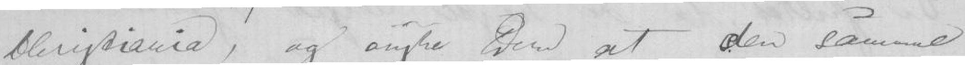Christiania, og ønske Dem at den samme
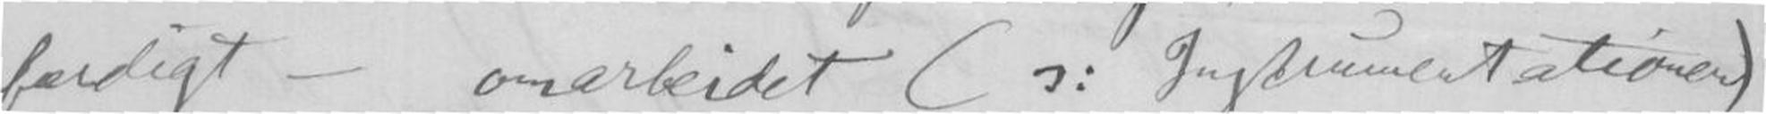færdigt - omarbeidet ( ): Instrumentationen)
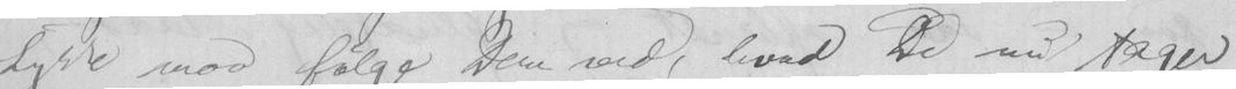Lykke maa følge Dem ved, hvad De nu tager
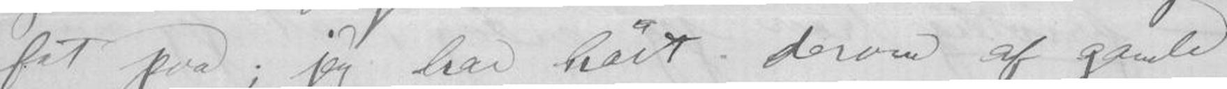fat paa; jeg har hørt derom af gamle
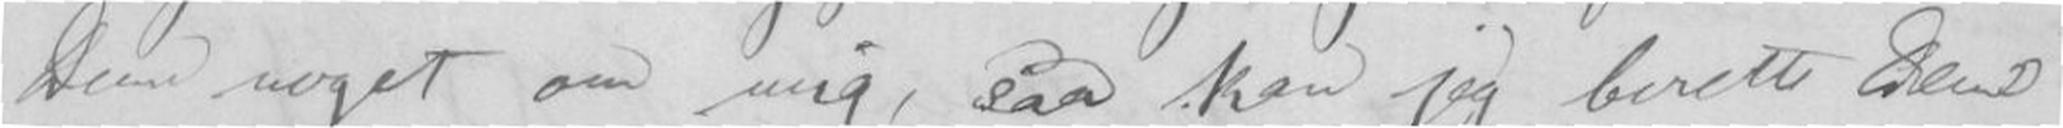Dem noget om mig, saa kan jeg berette Dem
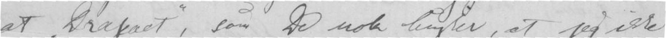at Drapoet, som De nok husker, at jeg ikke
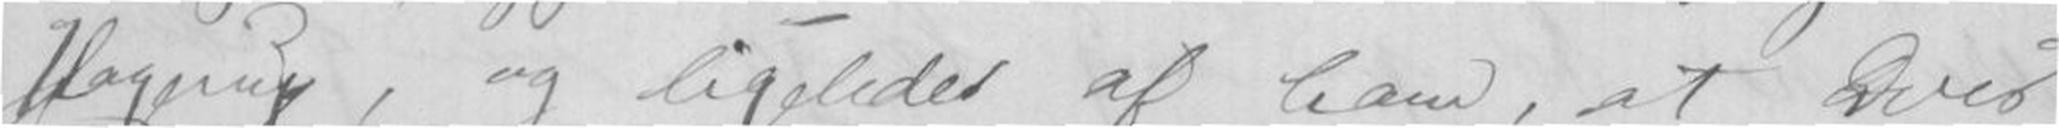Hagerup, og ligeledes af ham, at Deres
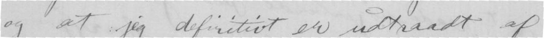og at jeg definitivt er udtraadt af
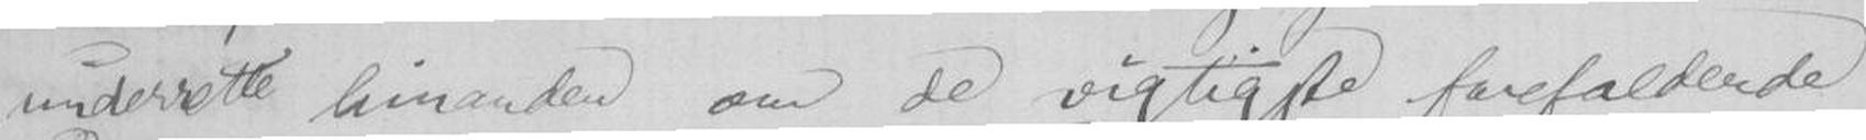underrette hinanden om de vigtigste forefaldende
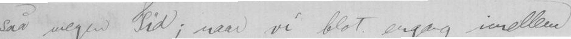saa megen Tid; naar vi blot engang imellem
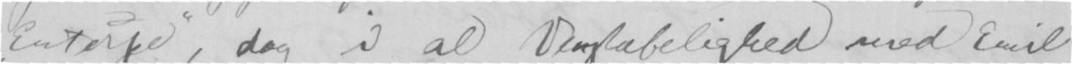Euterpe, dog i al Venskalbelighed med Emil
